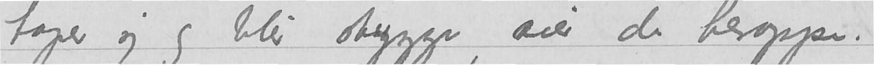taper sig og blir stygge, sier de heroppe.
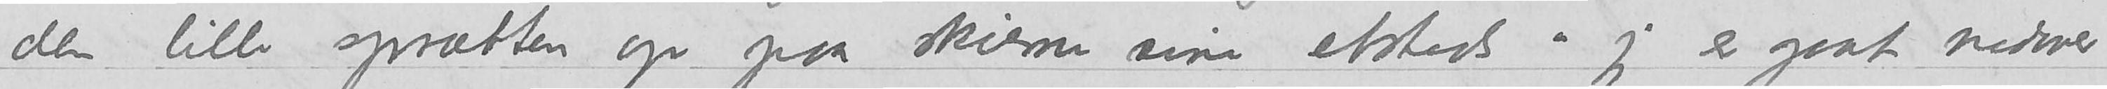den lille sprætten op paa skierne sine etsteds «jeg er gaat nedover
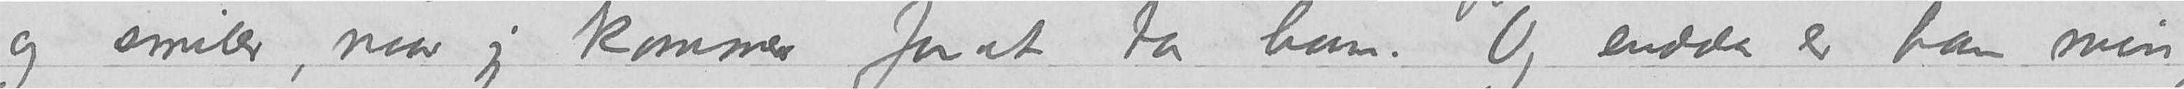og smiler, naar jeg kommer for at ta ham.  Og endda er han min,
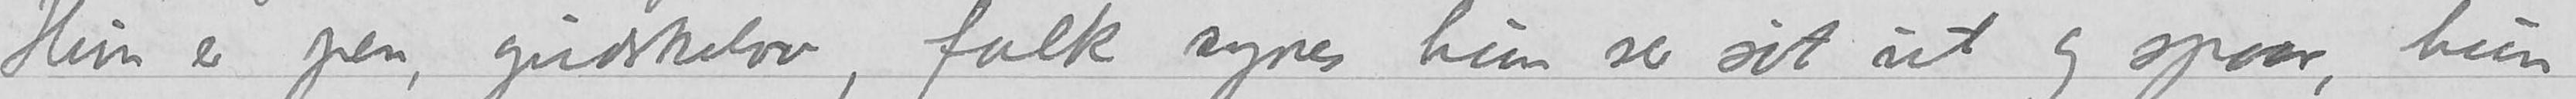Hun er pen, gudskelov, folk synes hun ser søt ut og spaar, hun
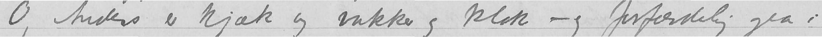Og Anders er kjæk og vakker og klok – og forfærdelig gla i
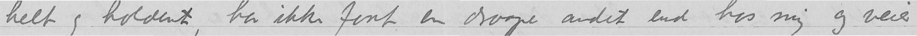helt og holdent, har ikke faat en draape andet end hos mig og veier
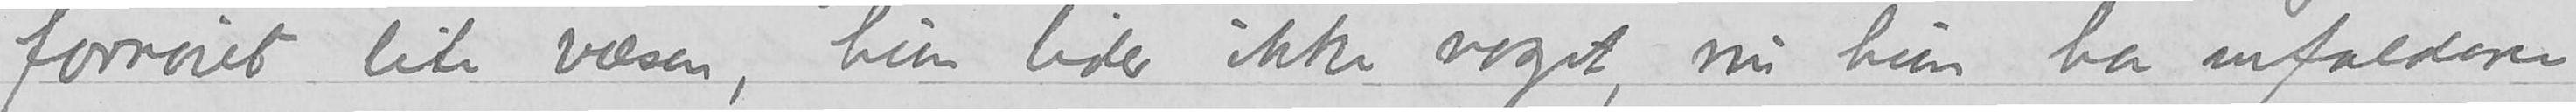fornøiet lite væsen, hun lider ikke noget, nu hun har anfaldene
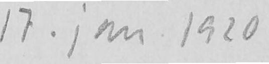17. Jan 1920
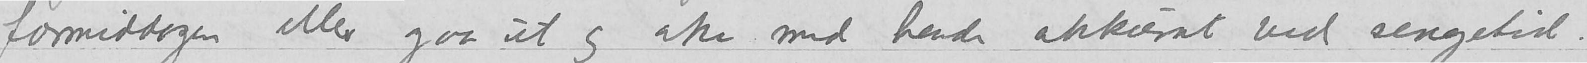formiddagen eller gaa ut og ake med hende akkurat ved sengetid.
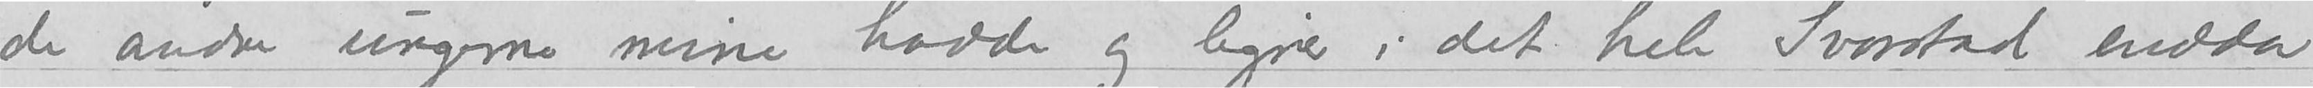de andre ungerne mine hadde og ligner i det hele Svarstad endda
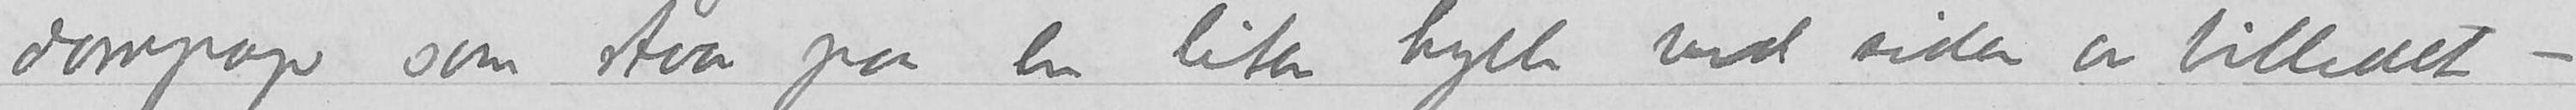dompap som staar paa en liten hylle ved siden av billedet –
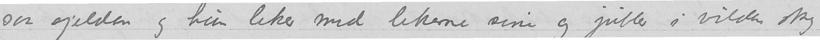saa sjelden og hun leker med lekerne sine og jubler i vilden sky
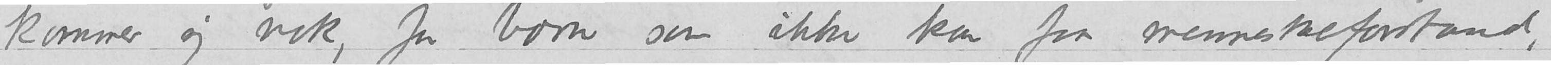kommer sig nok, for barn som ikke kan faa menneskeforstand,
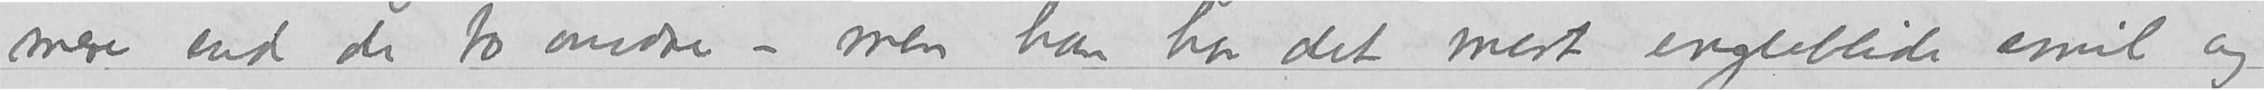mere end de to andre – men han har det mest engleblide smil og
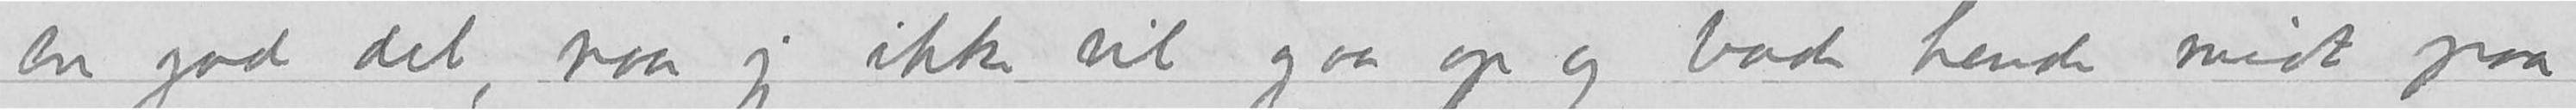hun en god del, naar jeg ikke vil gaa op og bade hende midt paa
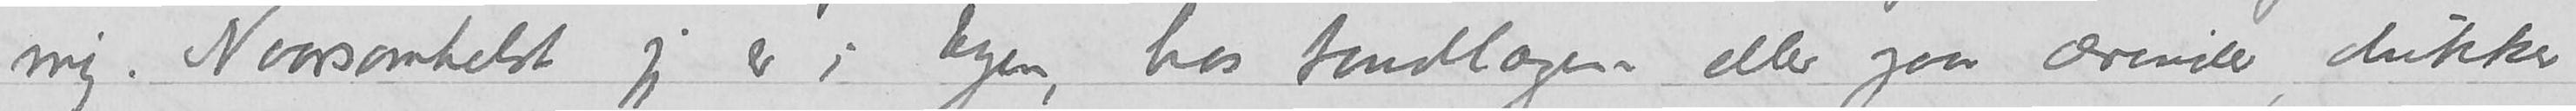mig. Naaar somhelst jeg er i byen, hos tandlægen eller gaar æriender, dukker
